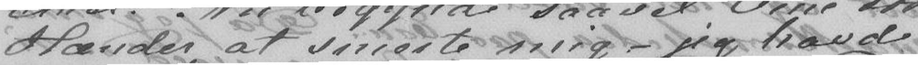Hænder at smerte mig - jeg havde
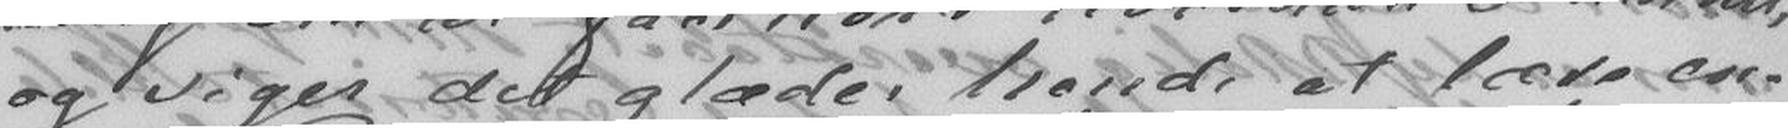og siger det glæder hende at læse om
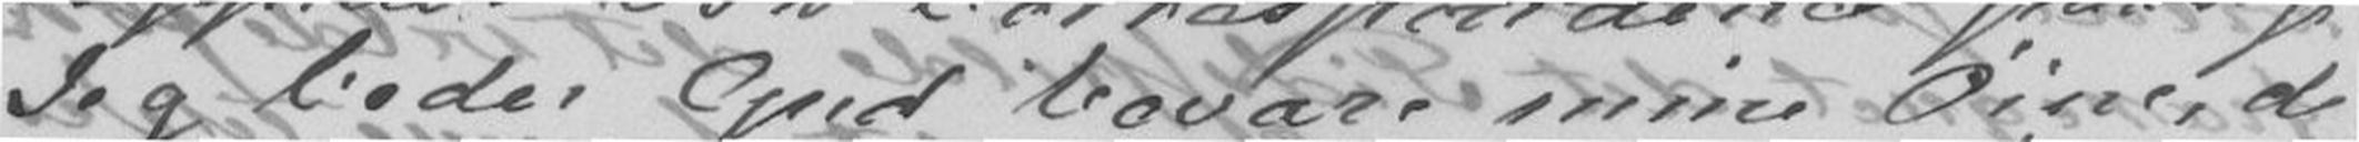Jeg beder Gud bevare mine Øine, de
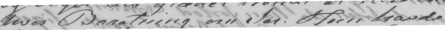hver Beretning om Jer. Hun havde
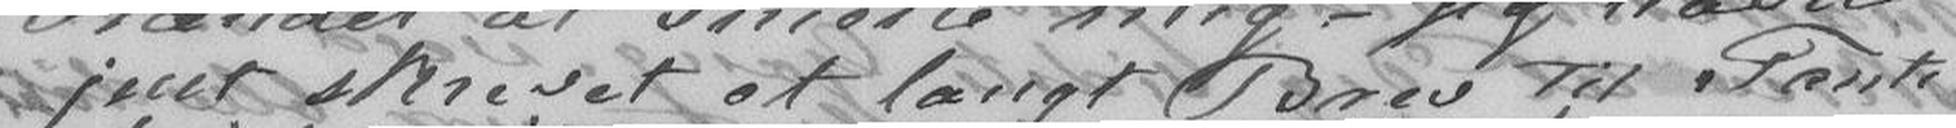just skrevet et langt Brev til Tante
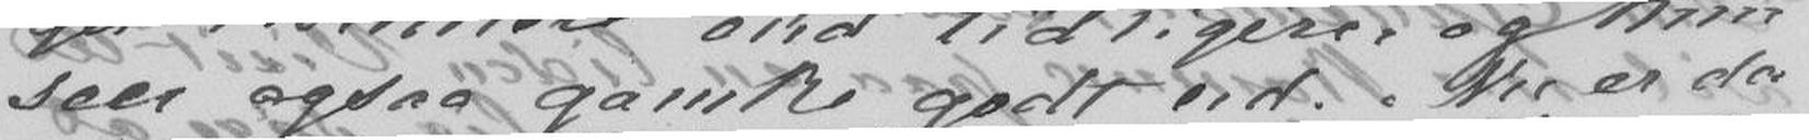seer ogsaa ganske godt ud. Nu er da
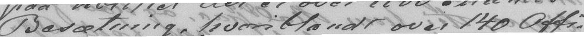Besætning, hvorblandt over 140 Offi-
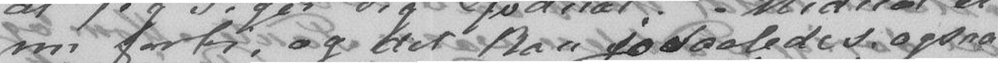nu forbi, og det kan jo saledes ogsaa
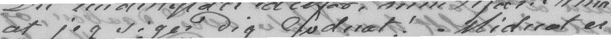at jeg siger Dig Godnat! Midnat er
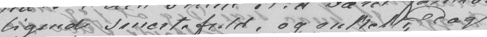ligend smertefuld, og enkelte Dag
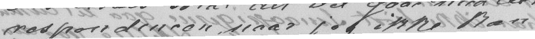respondecen naar jeg ikke kan
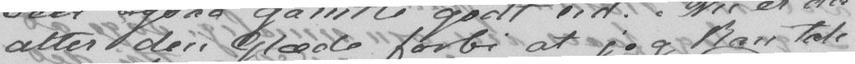atter den glæde forbi at jeg kan tale
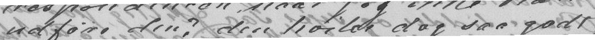udføre den? den hviler dog saa godt
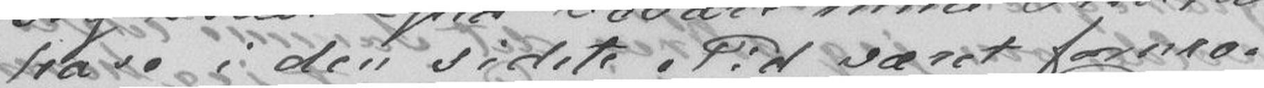have i den sidste Tid været foruro-
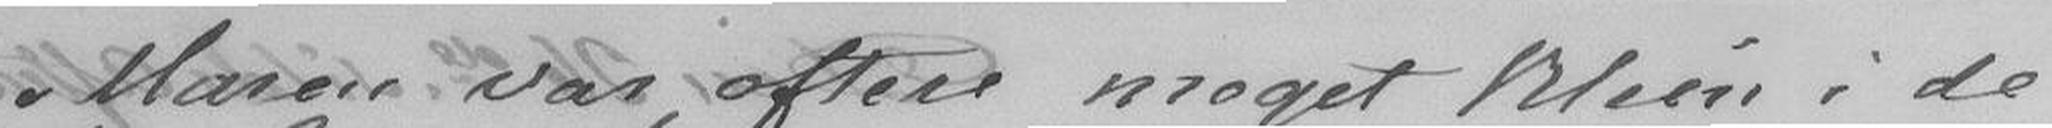Maren var oftere meget klein i de
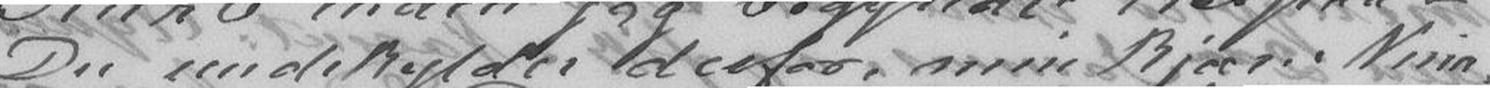Du undskylder derfor, min kjære Nina
